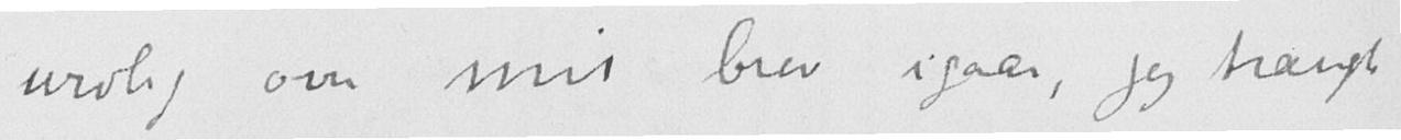urolig over mit brev i gaar, jeg trængte
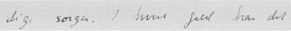dige sorger. I hvert fald har det
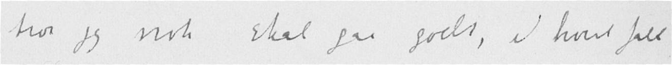tror jeg nok skal gaa godt, i hvert fald
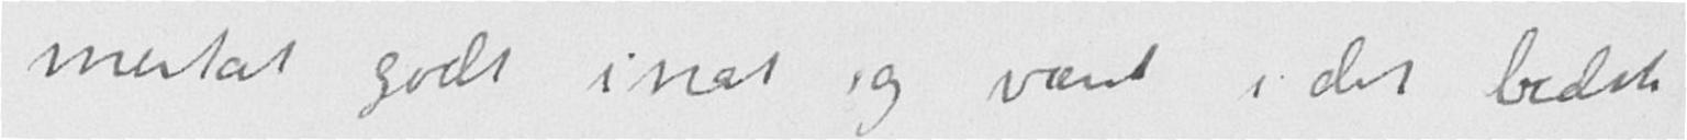merket godt i nat og været i det bedste
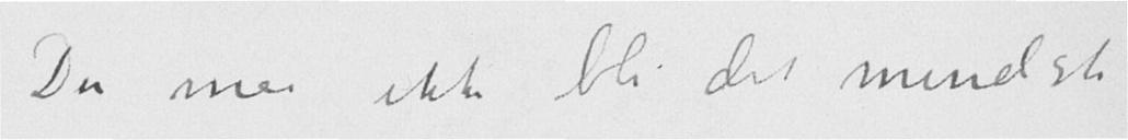Du maa ikke bli det mindste
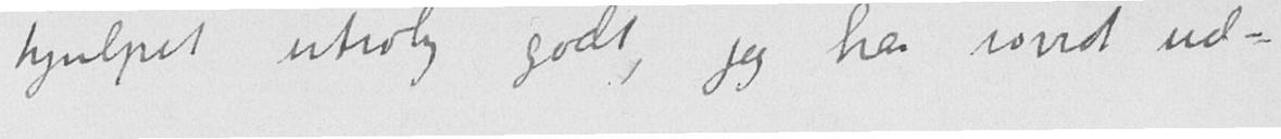hjulpet utrolig godt, jeg har sovet ud-
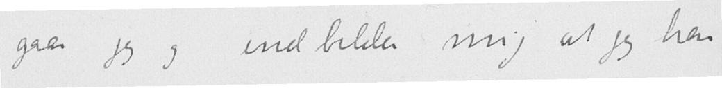gaar jeg og indbilder mig at jeg har
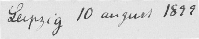Leipzig 10 august 1899
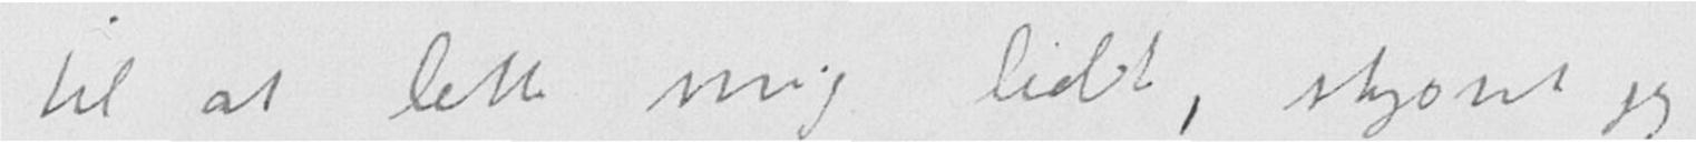til at lette mig lidt, skjønt jeg
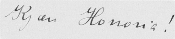Kjære Honoria!
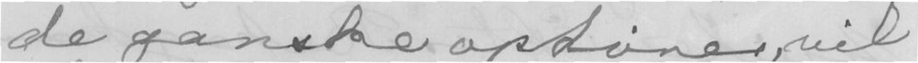de ganske ophører, vil
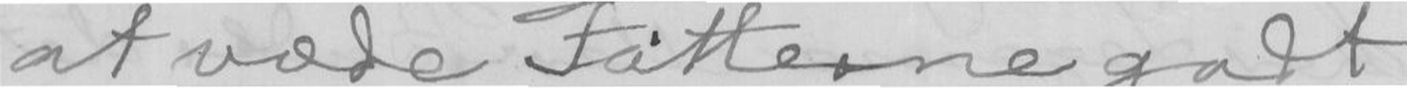at væde Føtterne godt
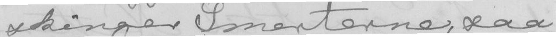skinger Smerterne, saa
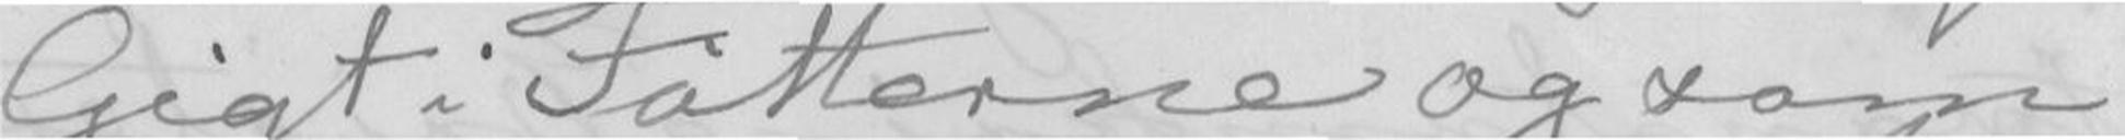Gigt i Føtterne og som
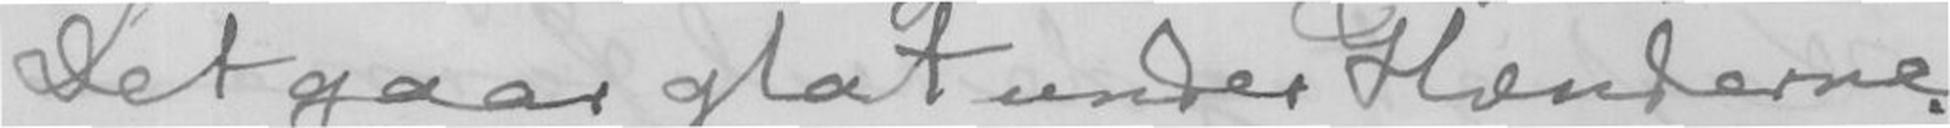Det gaar glat under Hænderne.
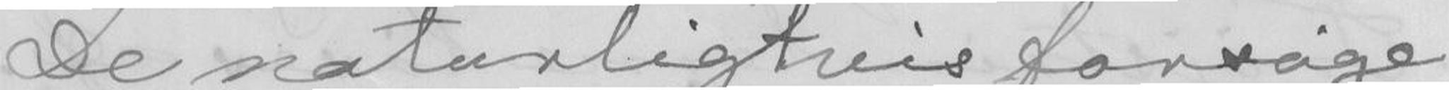De naturligtvis forsöge
